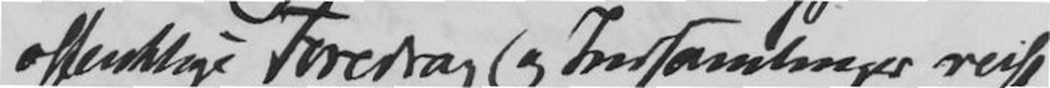offentlige Foredrag og Indsamlinger reise
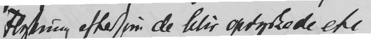Flytning efter som de blir opdyrkede etc.
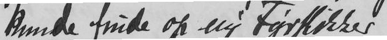kunde finde op ny Fyrstikker.
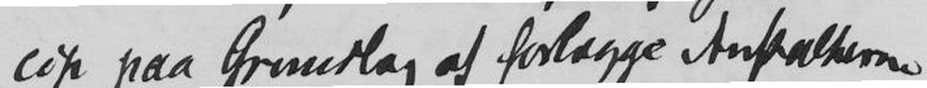cip paa Grundlag af forlægge Anstalterne
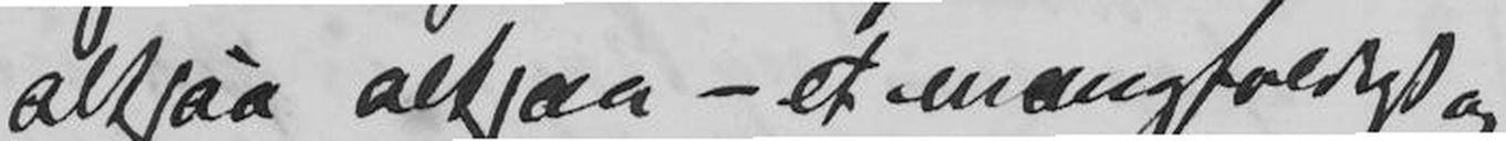altsaa altsaa - et mangfoldigt og
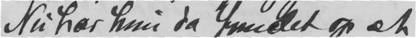Nu har hun da fundet op et
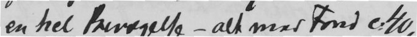en hel Bevægelse - alt med Fond c: 40,
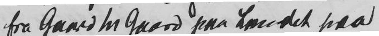fra Gaard til Gaard paa Landet paa
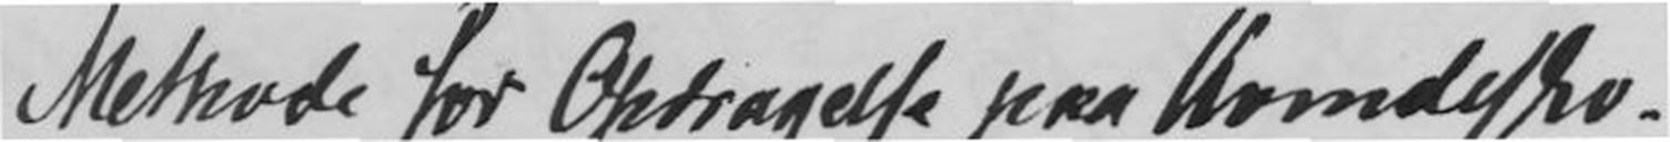Methode for Opdragelse paa Handelssko-
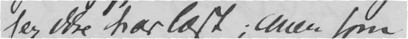jeg ikke har læst; men som
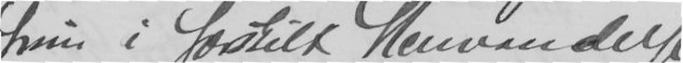hun i særskilt Henvendelse
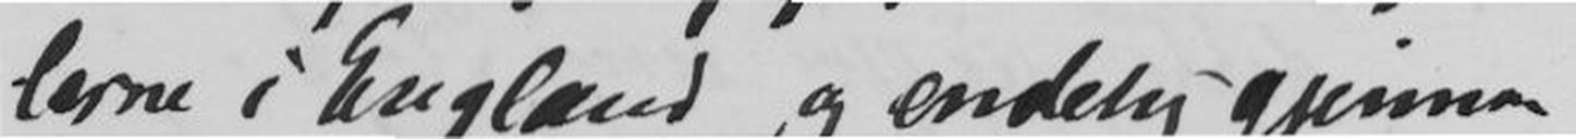lerne i England og endelig gjennem
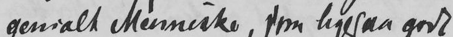genialt Menneske, som ligesaa godt
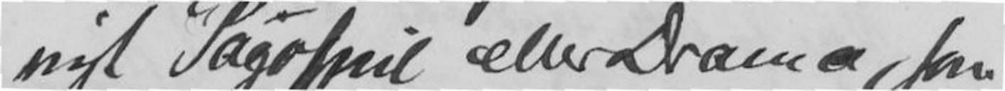nyt Sagospil eller Drama, som
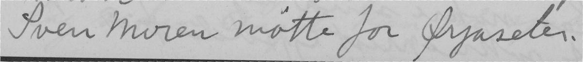Sven Moren mötte for Ørjaseter.
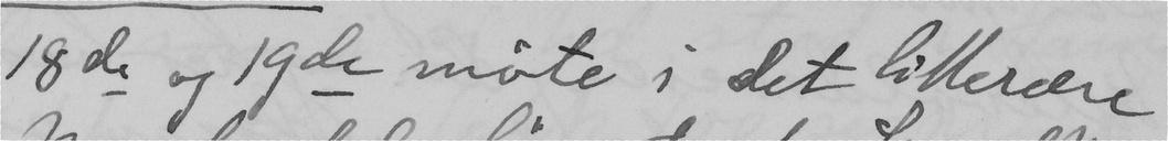18de og 19de möte i det litterære
